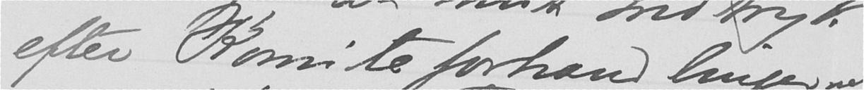efter Komiteforhandlingerne
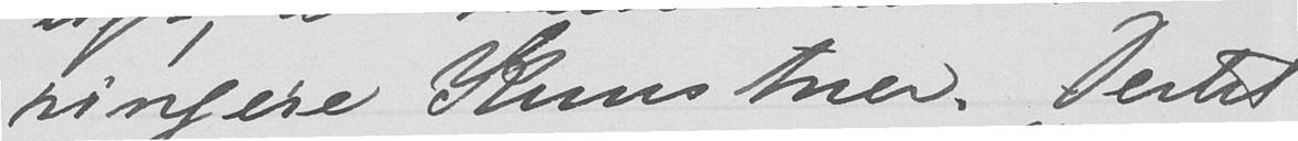ringere Kunstner. Dertil
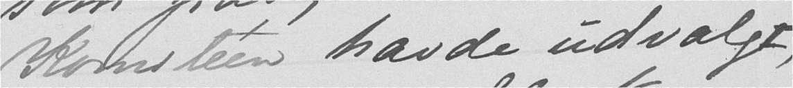Komitéen havde udvalgt,
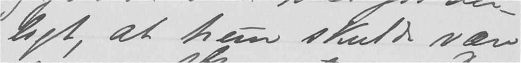ligt, at hun skulde være
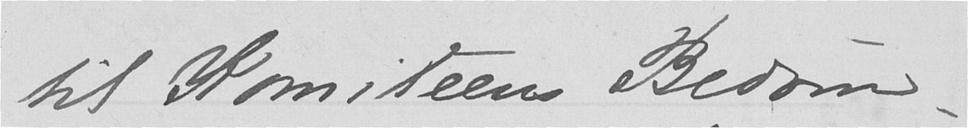til Komiteens Bedøm-
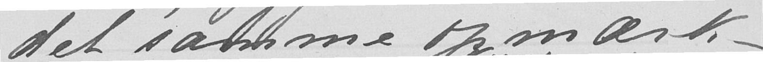det samme opmærk-
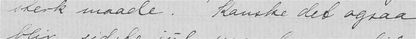sterk maade. Kanske det ogsaa
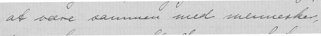at være sammen med mennesker,
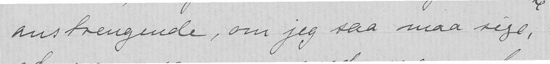anstrengende, om jeg saa maa sige,
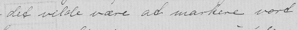- det vilde være at markere vort
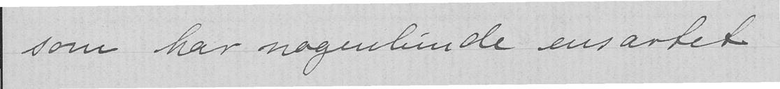som har nogenlunde ensartet
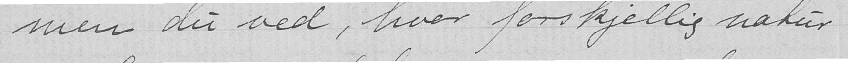men du ved, hvor forskjellig natur
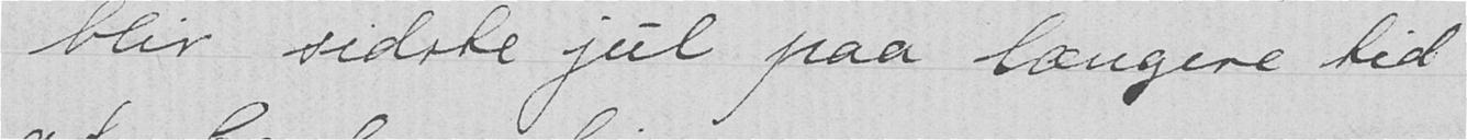blir sidste jul paa længere tid
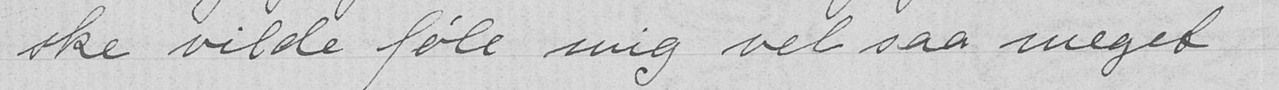ske vilde føle mig vel saa meget
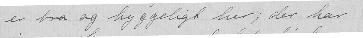er bra og hyggeligt her; der har
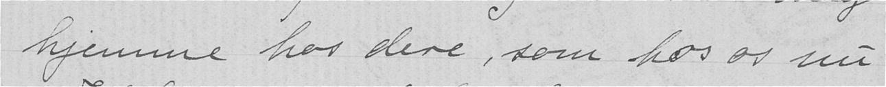hjemme hos dere, som hos os nu.
